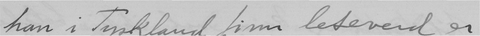han i Tyskland finn leseverd er
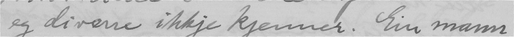eg diverre ikkje kjenner. Ein mann
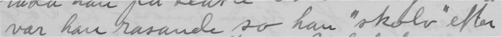var han rasande so han "skolv" etter
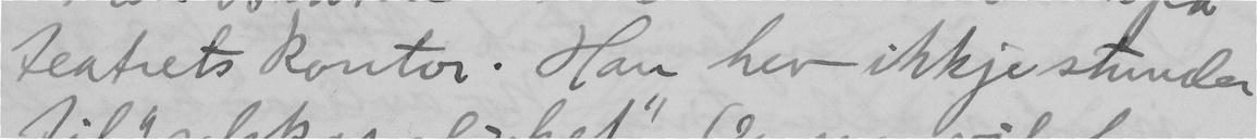teatrets kontor. Han hev ikkje stunder
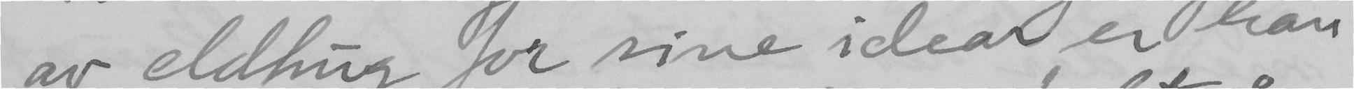av eldhug for sine idear er han
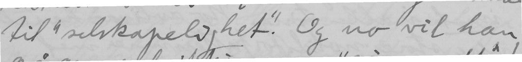til "selskapelighet". Og no vil han
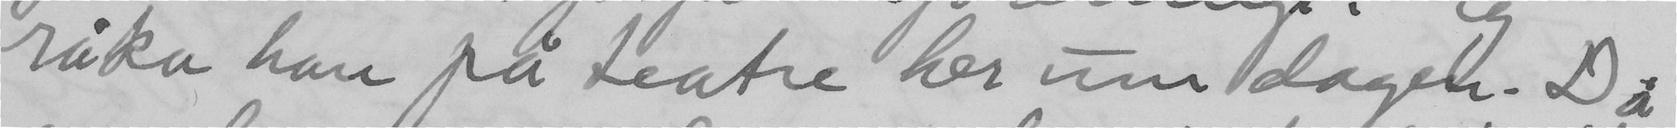råka han på teatre her um dagen. Då
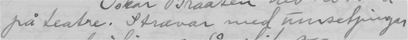på teatre. Strævar med umsesetjingar
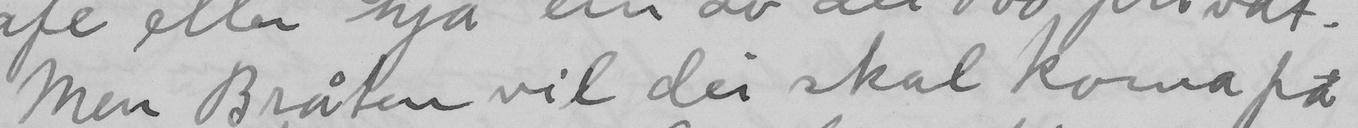Men Bråten vil dei skal koma på
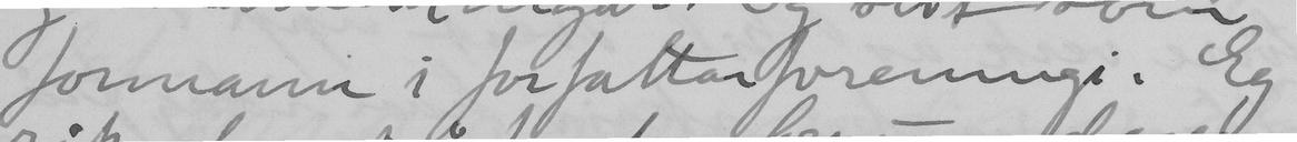formann i fortatterforeningi. Eg
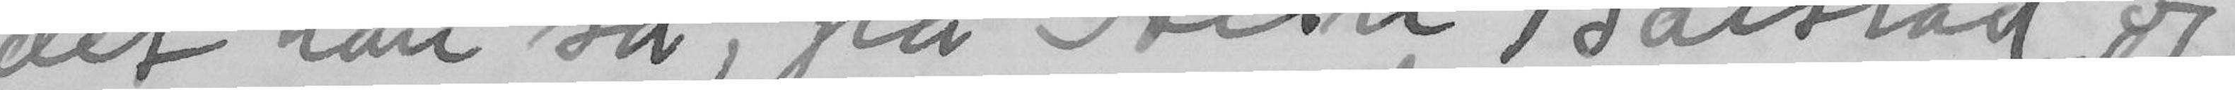det han, på Stein Balstad og
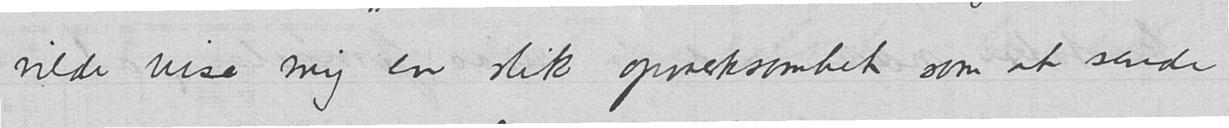vilde vise mig en slik opmerksomhet som at sende
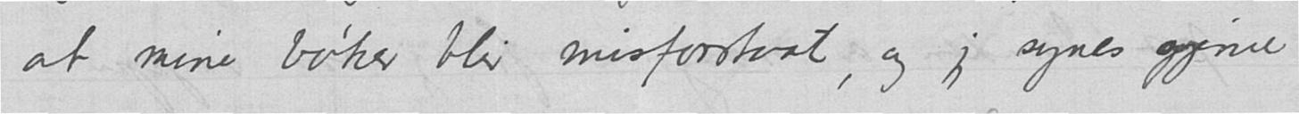at mine bøker blir misforstaat, og jeg synes gjerne
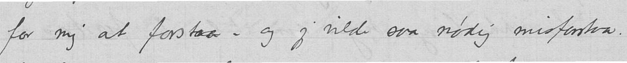for mig at forstaa – og jeg vilde saa nødig misforstaa.
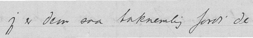jeg er Dem saa taknemlig fordi De
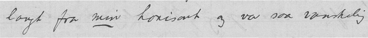langt fra min horisont og var saa vanskelig
